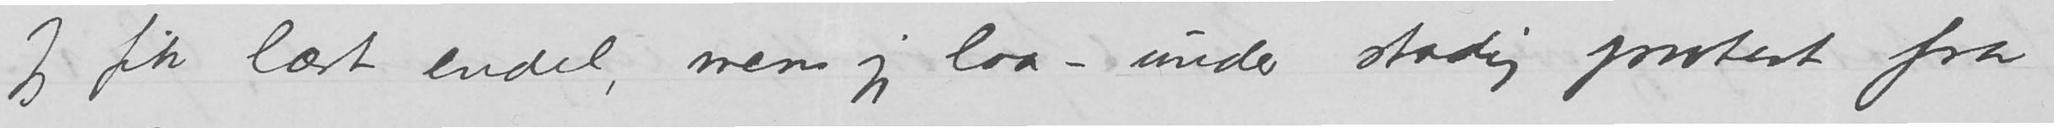Jeg fik læst endel, mens jeg laa – under stadig protest fra
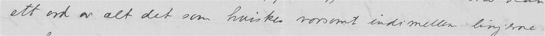ett ord av alt det som hviskes varsomt indimellem linjerne
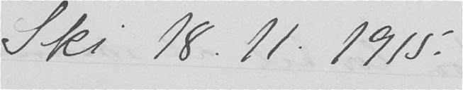Ski 18.11. 1915.
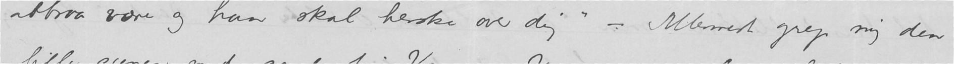attraa være og han skal herske over dig» – Allermest grep mig den
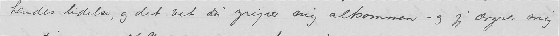hendes lidelse, og det vet du griper mig altsammen – og jeg ærgrer mig
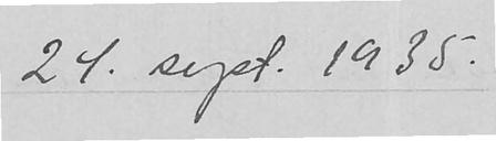24. sept. 1935.
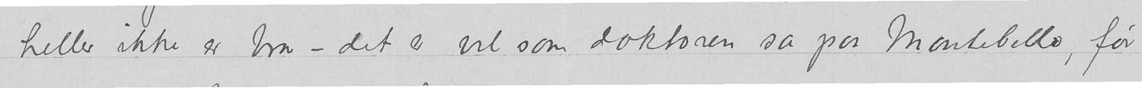heller ikke er bra – det er vel som doktoren sa paa Montebello, før
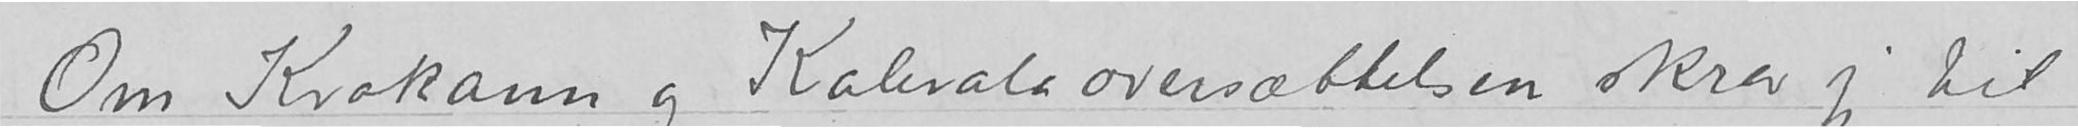Om Krokann og Kalevalaoversættelsen skrev jeg til
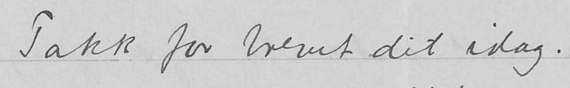Takk for brevet dit idag.
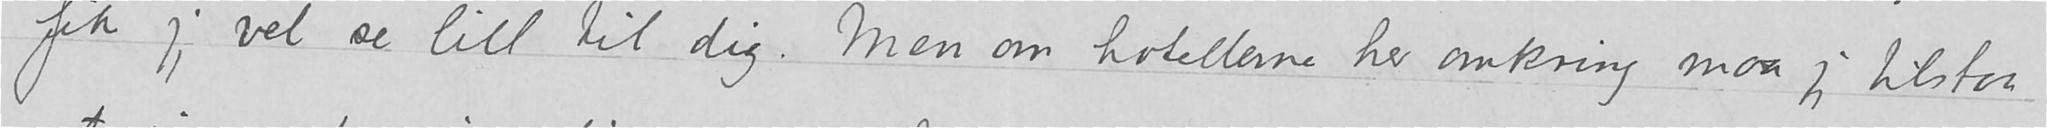fik jeg vel se litt til dig. Men om hotellerne her omkring maa jeg tilstaa
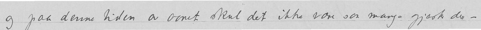og paa denne tiden av aaret skal det ikke være saa mange gjester der –
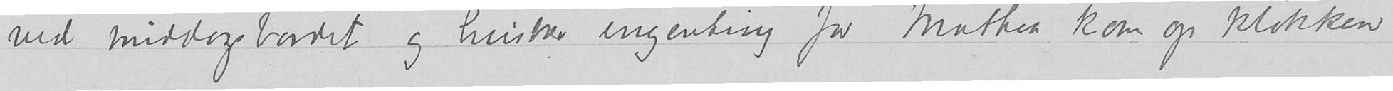ved middagsbordet og husker ingenting for Mathea kom op klokken
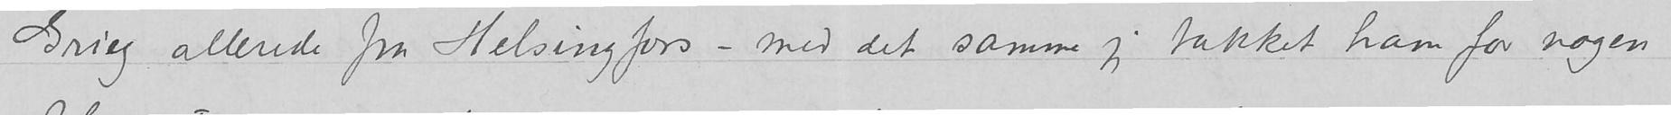Grieg allerede fra Helsingfors – med det samme jeg takket ham for nogen
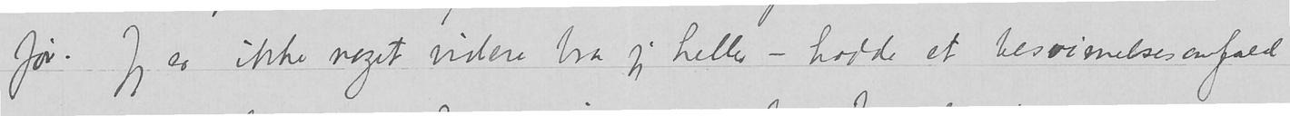før. Jeg er ikke noget videre bra jeg heller - hadde et besvismelsesanfald
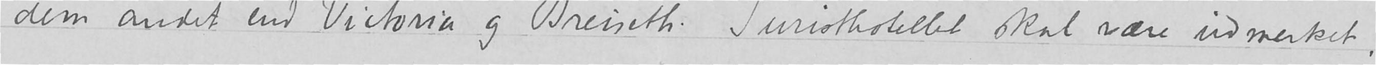den andet end Victoria og Breiseth. Turisthotellet skal være udmerket,
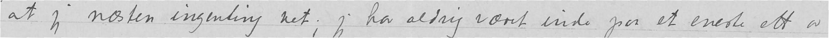at jeg næsten ingenting vet; jeg har aldrig været inde paa et eneste ett av
